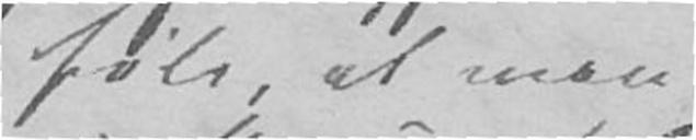føle, at man
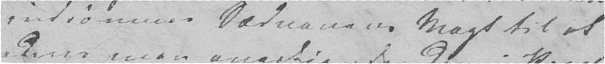indrømme Sædvanen Magt til at
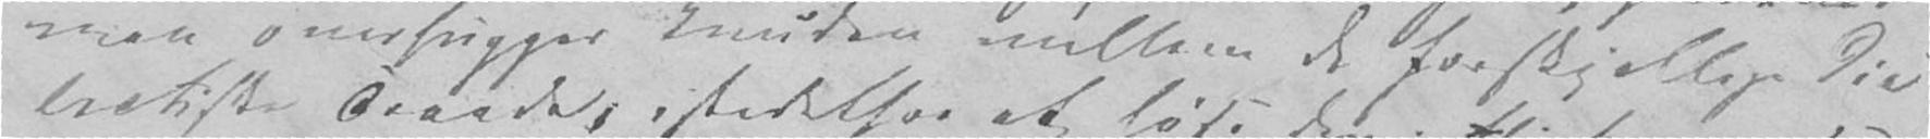man overhugger Knuden mellem de forskjellige dia-
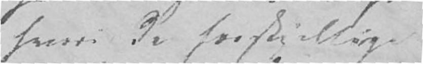hvori de forskjellige
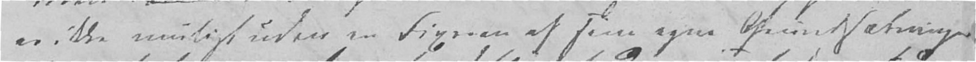er ikke muligt uden en Fixeren af sine egne Grundsætninger
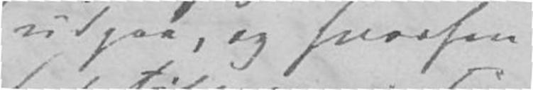udgaa, og hvorfra
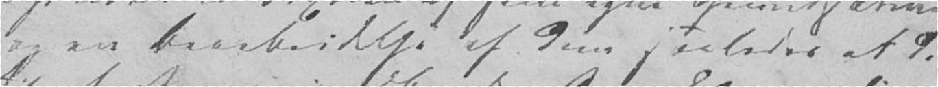og en Bearbeidelse af dem saaledes at de
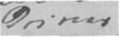drives
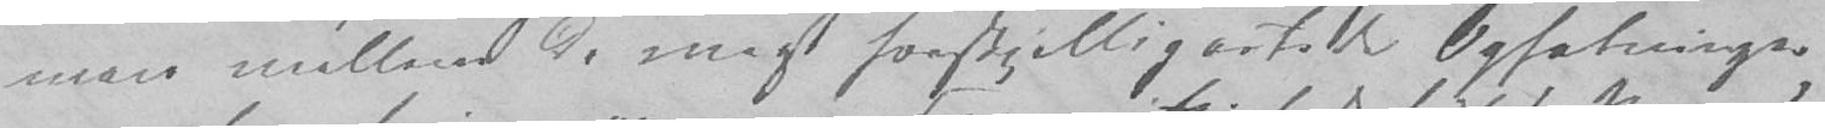man mellem de mest forskjelligartede Opfatninger
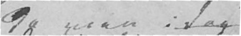Da man i dag
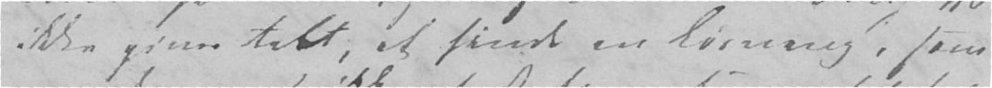ikke giver tabt, at finde en Løsning, som
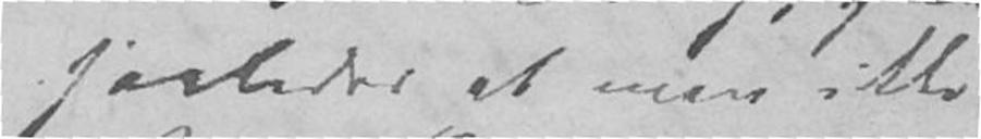saaledes at man ikke
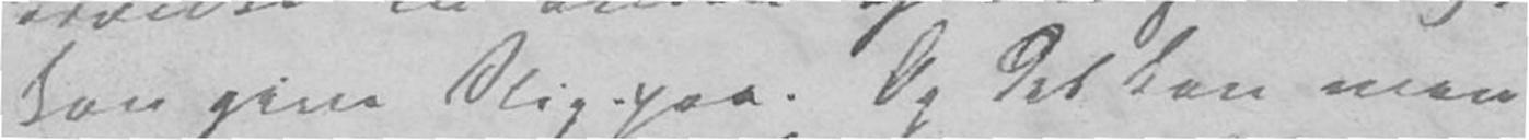kan give Slip paa. Og det kan man
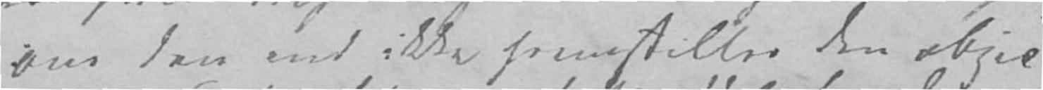om den end ikke fremstiller den objec-
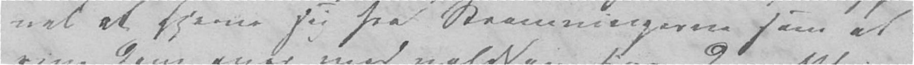vel at fjerne sig fra Strømningerne som at
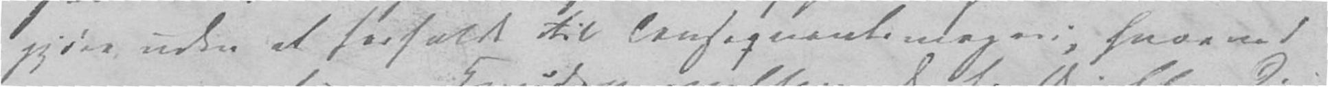gjøre uden at forfalde til Conseqventsmageri, hvorved
